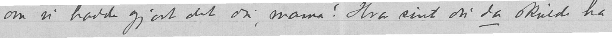om vi hadde gjort det du, mama! Hvor sint du da skulde ha
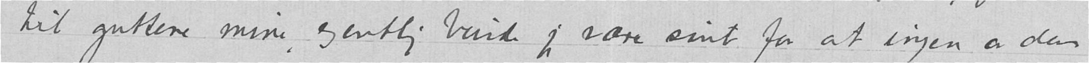til guttene mine, egentlig burde jeg være sint for at ingen av dem
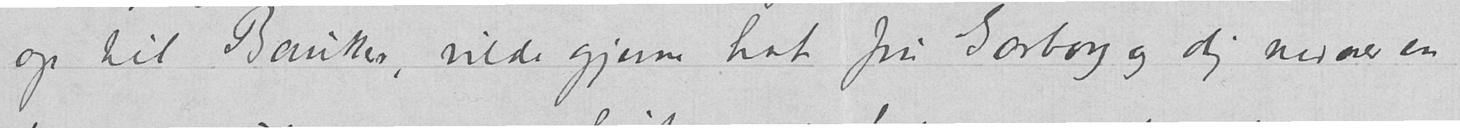op til Bauker, vilde gjerne hat fru Garborg og dig nedover en
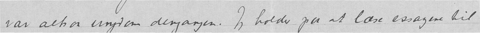var altsaa ungdom dengangen. Jeg holder paa at læse essayene til
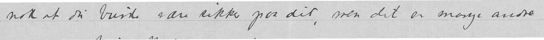nok at du burde være sikker paa dit, men det er mange andre
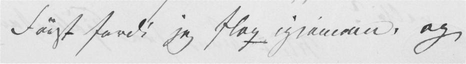Først fordi jeg slap igjennem, og
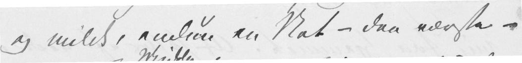og mildt, endnu en Nat – den værste –
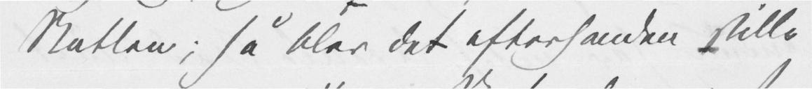Natten; så blev det efterhanden stille
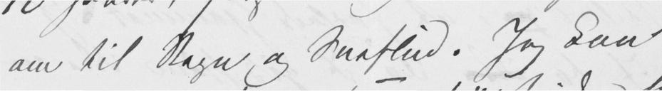om til Regn og Sneslud. Jeg kan
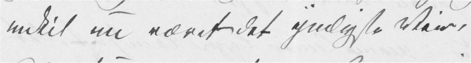indtil nu været det yndigste Veir,
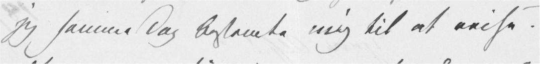jeg samme Dag bestemte mig til at reise.
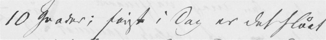10 Grader; først i Dag er det slået
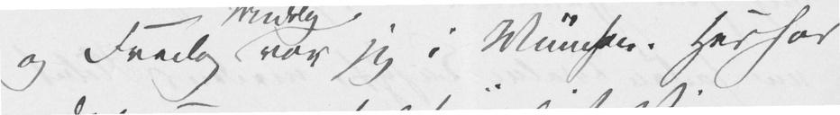og Fredag var jeg i München. Her har
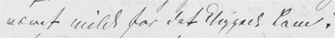været mild for det klippede Lam."
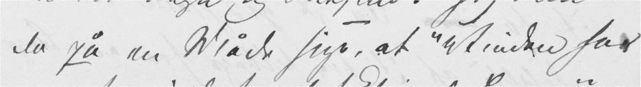da på en Måde sige, at "Vinden har
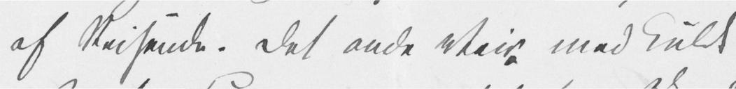af Reisende. Det onde Veir med Kulde
